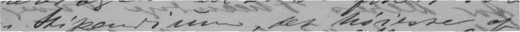i Stipendium, det høieste af
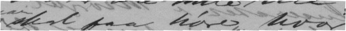skal faa høre hvor
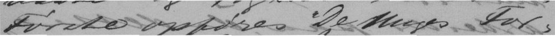Første opføres "De Unges For-
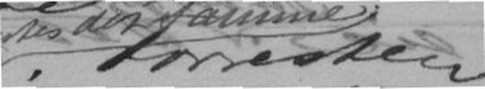, foresten
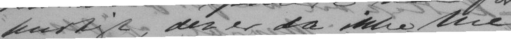hurtigt, der er da ikke Me-
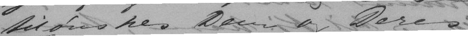til ønskes Dem og Deres
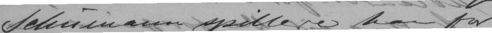Schumann spillede han for
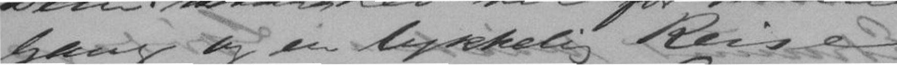Gang og en lykkelig Reise
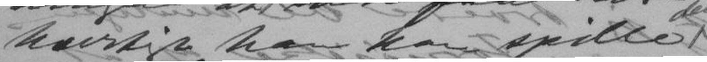hurtigt han kan spille
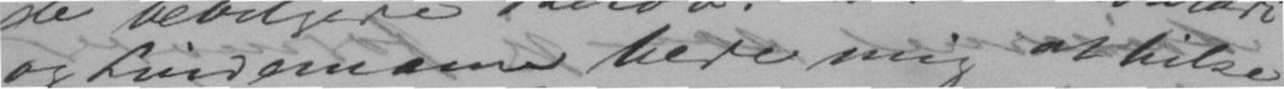og Lindemann bede mig at hilse
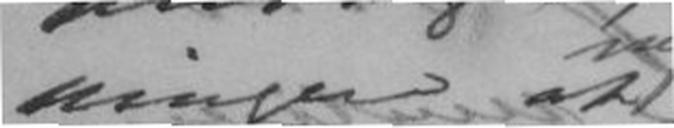ningen at
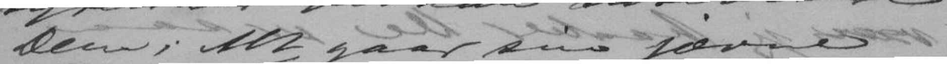Dem; Alt gaar sin jævne
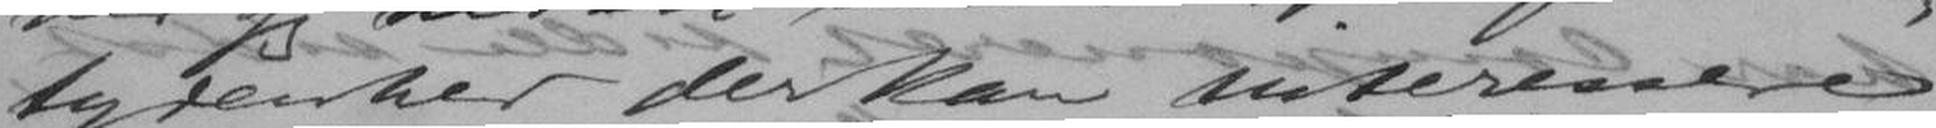tydenhed der kan interessere
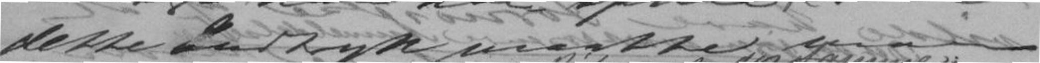dette Indtryk maatte man
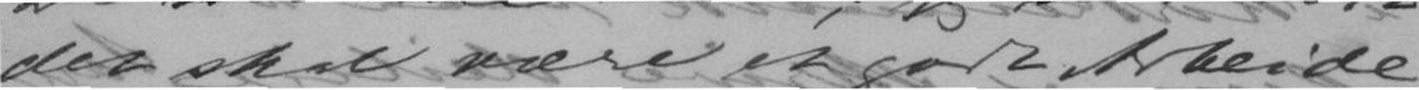det skal være et godt Arbeide,
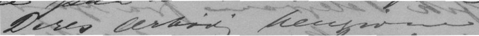Deres ærbødig hengivne
